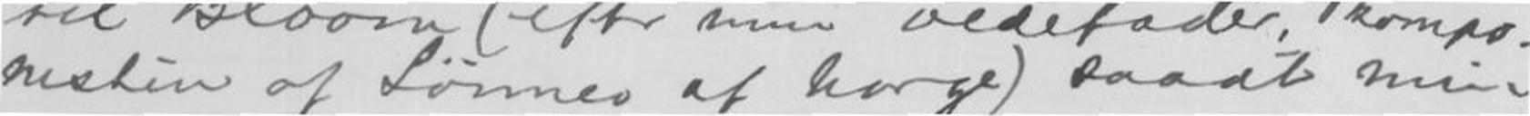nisten af Sønner af Norge) saaat min
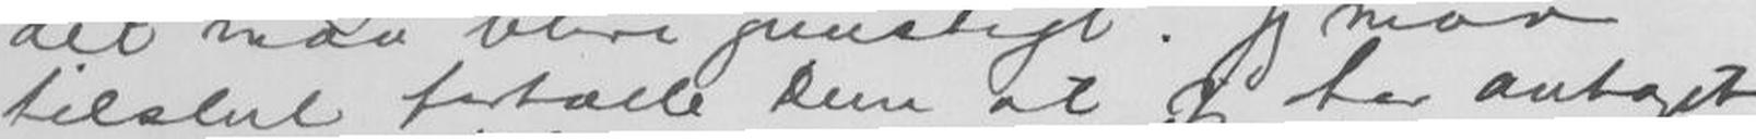tilslut fortælle Dem at jeg har antaget
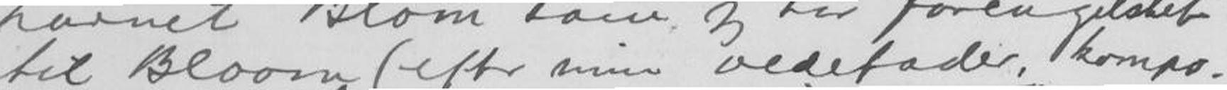til Bloom (efter min vedefader, kompo-
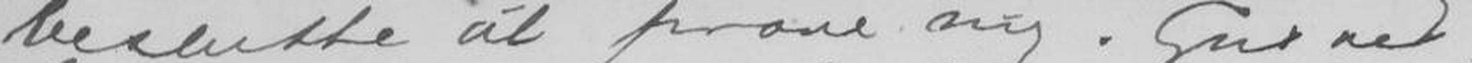beslutte at prøve mig. Gud ved
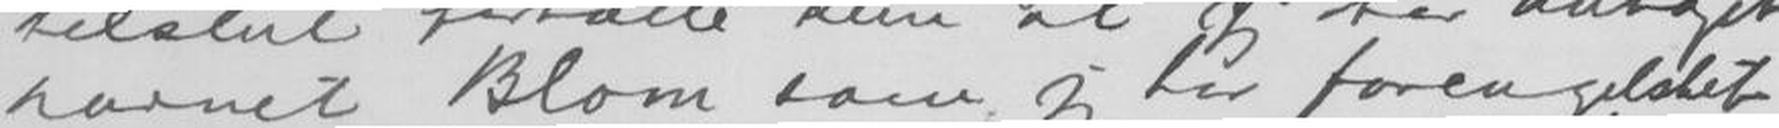Navnet Blom som jeg har forengelsket
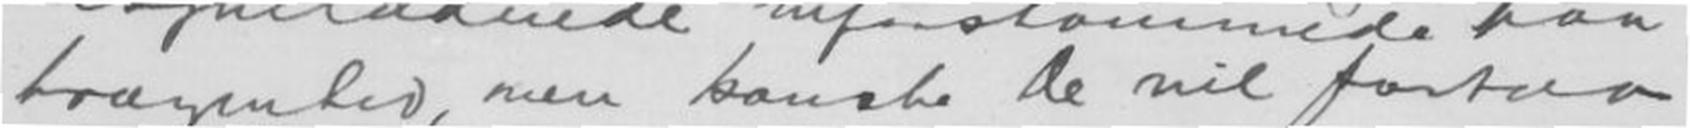trængenhed men kanske De vil forstaa
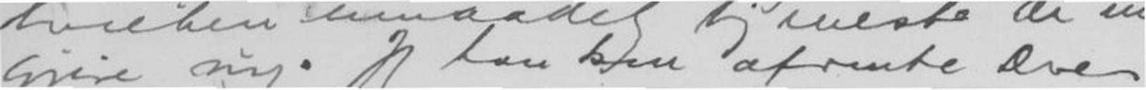Gjøre mig. Jeg kan kun afvente Deres
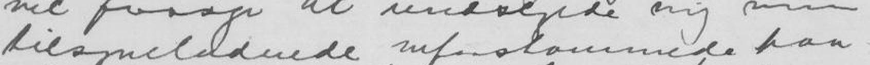tilsyneladende uforskammede paa-
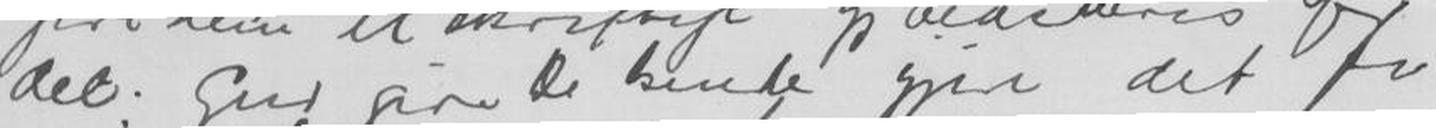det. Gud give De kunde gjøre det
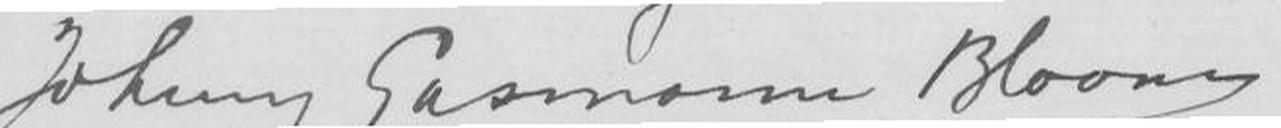Johnny Gasmann Bloom
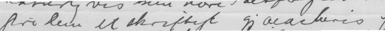give Dem et skriftligt gjældsbevis for
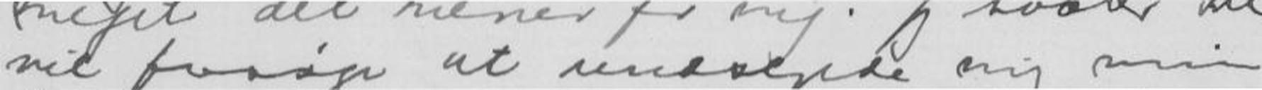vil forsøge at undskylde mig min
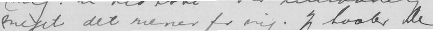meget det mener for mig. Jeg haaber De
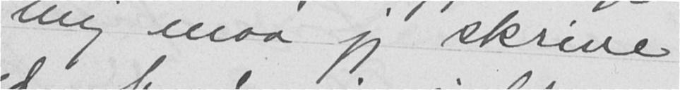mig maa jeg skrive
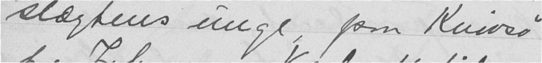slægtens unge, paa Knivsø
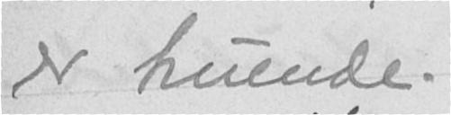er truende.
